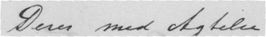Deres med Agtelse
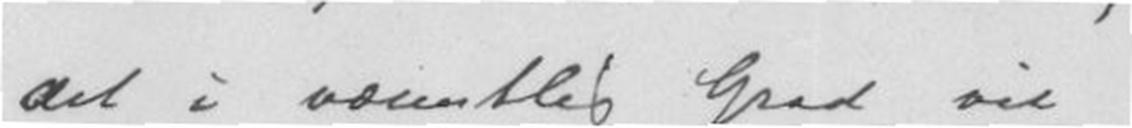del i uæentlig Grad vil
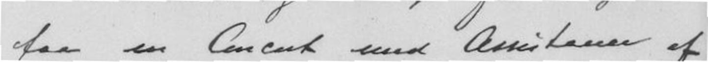faa en Concert med Assistansen af
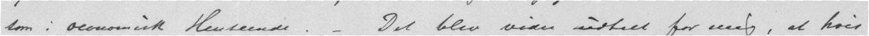som; Henseende.- Det blev videre udtalt for mig, at hvis
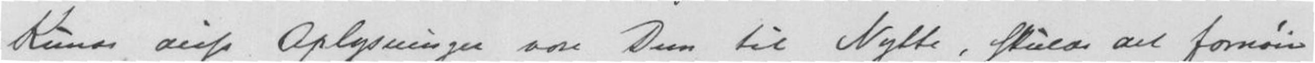Kunde disje Oplysninger være Dem til Nytte, Skulde det fornøie.
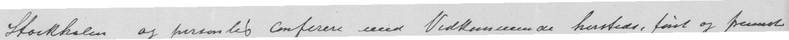Stockholm af personlig Conferere med Vedkommende , først og fremst
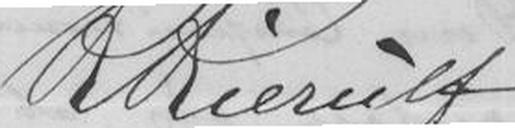RKierulf
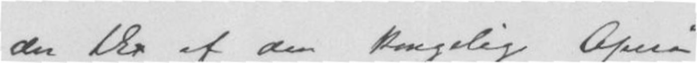der af den kongelig Opera
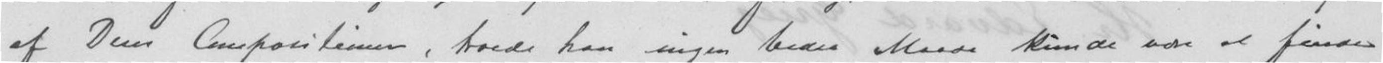af Dens Componitimer, troede han ingen bedre maade kunde være at findes
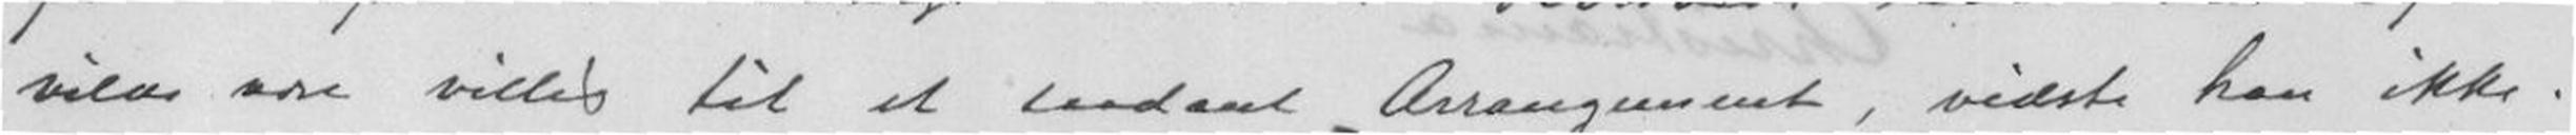vilde villig til et saadant Arrangement, vidste han ikke.
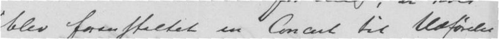blev foranltet en Concert til udførelse
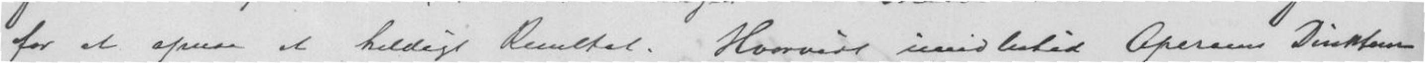for at opnaa et heldigt Resultat. Hvorvidt imidlertid Operaens Dim
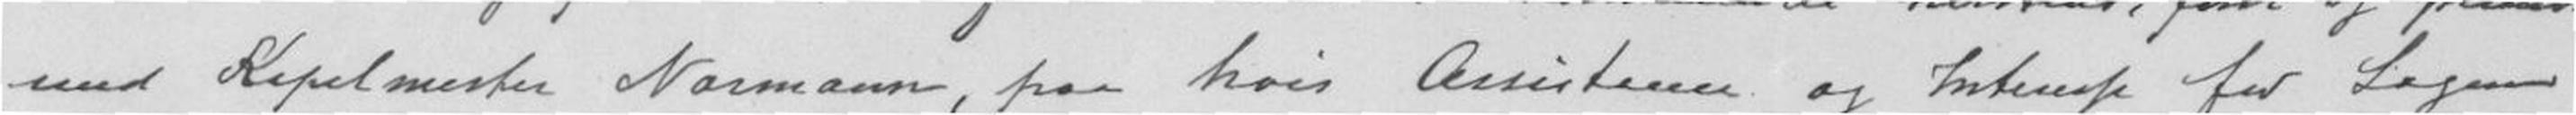med Kapelmester Normann, paa høis Assistanse og interesje for Sagene
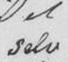selv
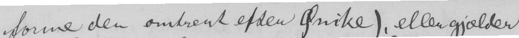forme den omtrnt efter Ønske), eller gjælder
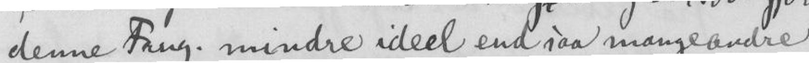denne Fang. mindre ideel end saa mange andre
